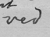ved
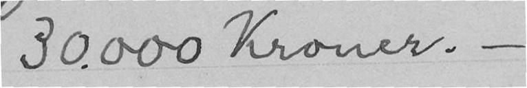30.000 Kroner. -
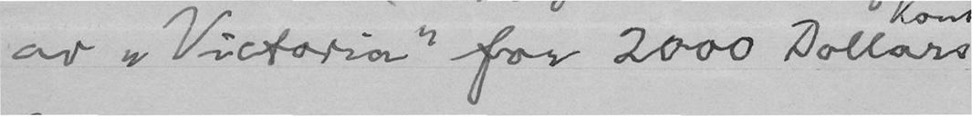av "Victoria" for 2000 Dollars
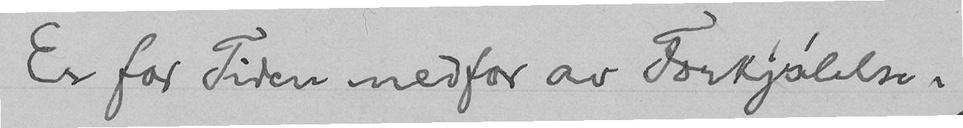Er for Tiden nedfor av Forkjølelse.
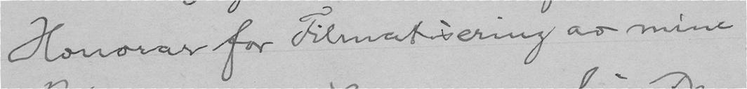Honorar for Filmatisering av mine
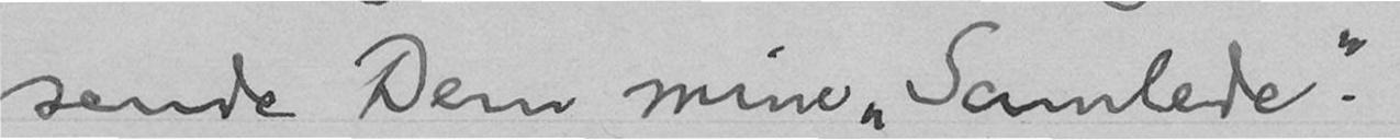sende Dem mine "Samlede".
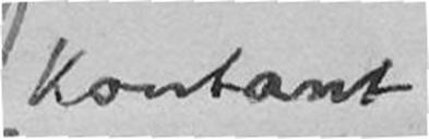kontant
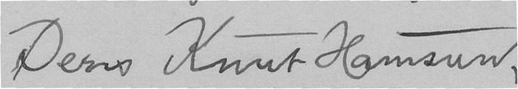Deres Knut Hamsun.
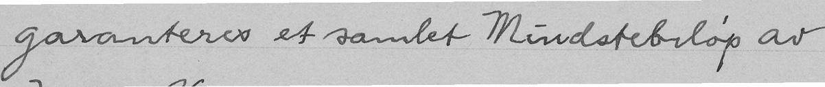garanteres et samlet Mindstebeløp av
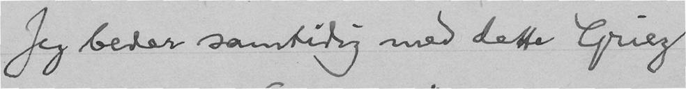Jeg beder samtidig med dette Grieg
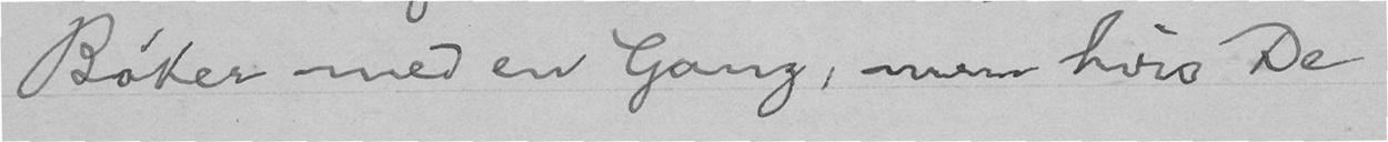Bøker med en Gang, men hvis De
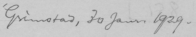Grimstad, 30 Januar 1929.
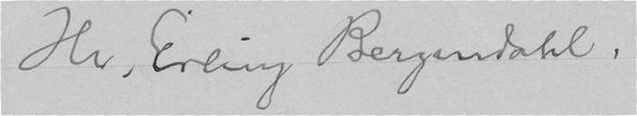Hr. Erling Bergendahl.
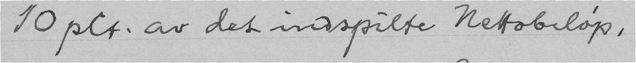10 pct. av det indspilte Nettobeløp,
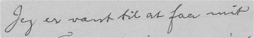Jeg er vant til at faa mit
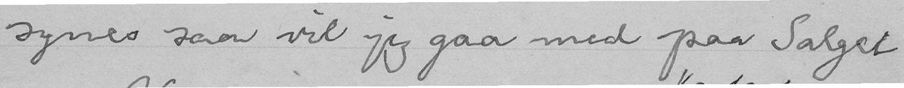synes saa vil jeg gaa med paa Salget
